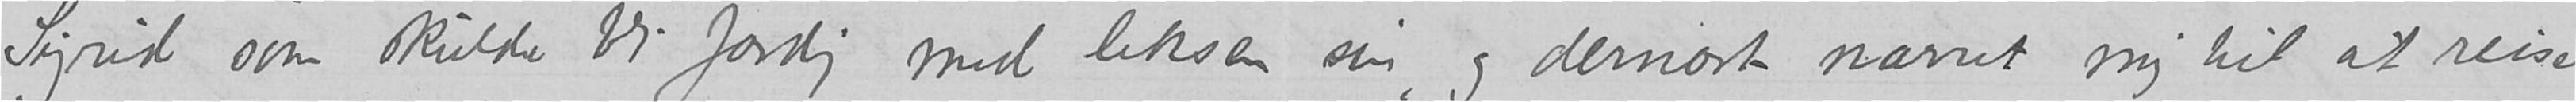Sigrid som skulde bli færdig med leksen sin, og dernæst narret mig til at reise
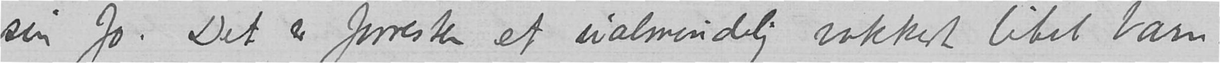sin Jo. Det er forresten et ualmindelig vakkert litet barn.
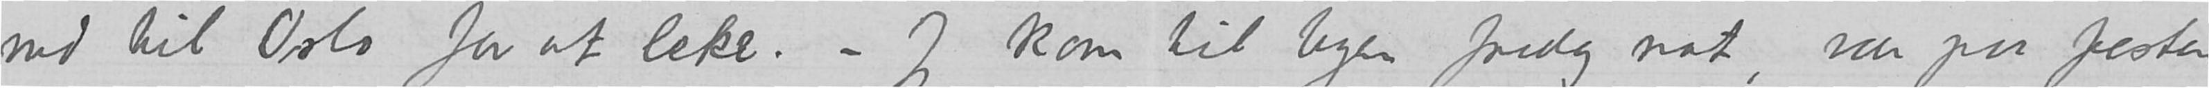ned til Oslo for at leke. – Jeg kom til byen fredag nat, var paa festen
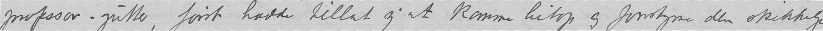professor-gutter, først hadde tillatt sig at komme hitop og forstyrre den skikkelige
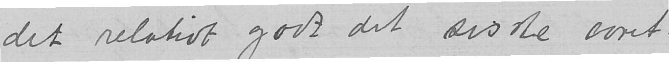det relativt godt det sisste aaret.
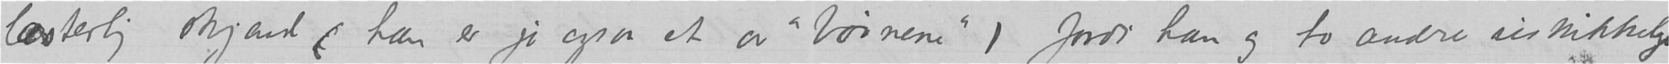lasterlig skjænd (han er jo ogsaa et av «børnene») fordi han og to andre uskikkelige
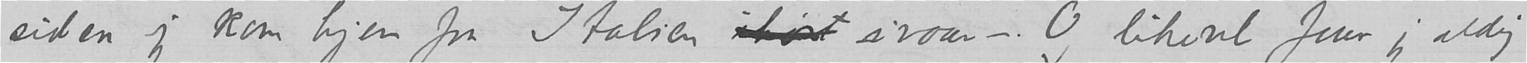siden jeg kom hjem fra Italien ihøst ivaar –. Og likevel faar jeg aldrig
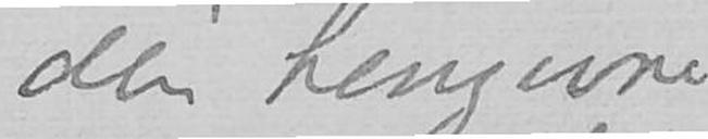din hengivne
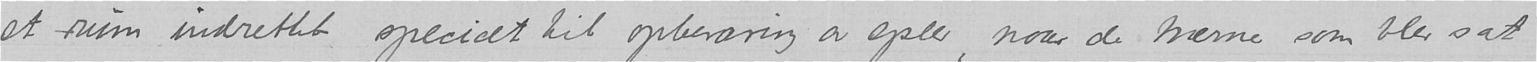et rum indrettet specielt til opbevaring av epler, naar de trærne som blev sat
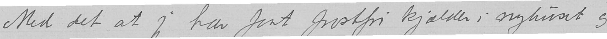Med det at jeg har faat frostfri kjælder i nyhuset og
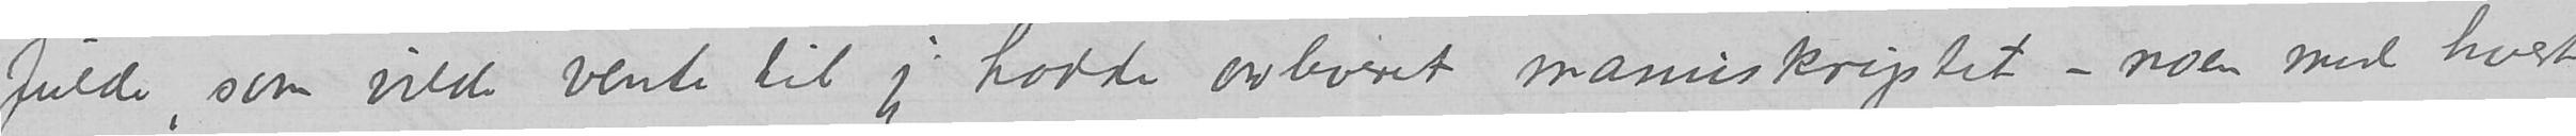fulde, som vilde vente til jeg hadde avleveret manuskriptet – noen med hvert
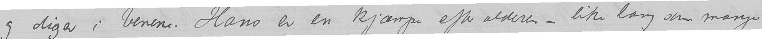og diger i benene. Hans er en kjæmpe efter alderen – like lang som mange
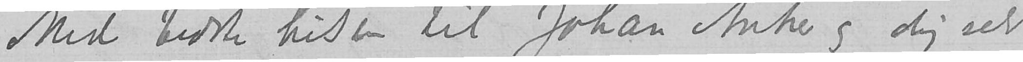Med bedste hilsen til Johan Anker og dig selv
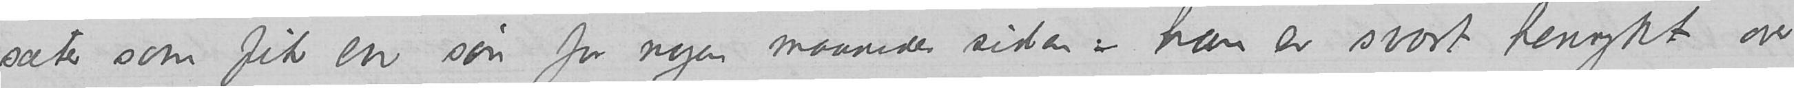sæter som fik en søn for nogen maaneder siden – han er svært henrykt over
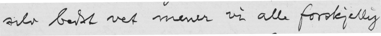selv bedst vet mener vi alle forskjellig
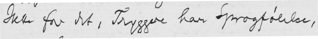Ikke for det, Tyggve har Sprogfølelse,
